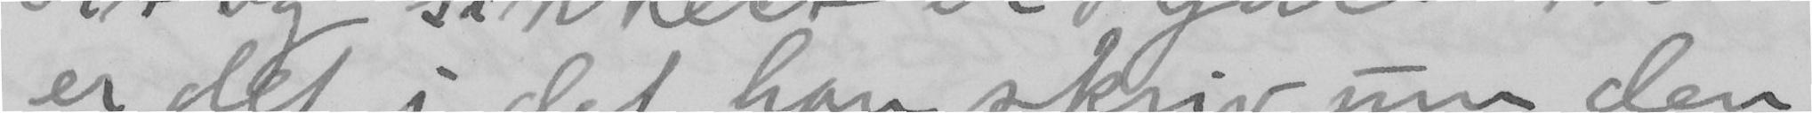er det i det han skriv um den
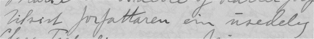tilsist forfattaren ein usedeleg
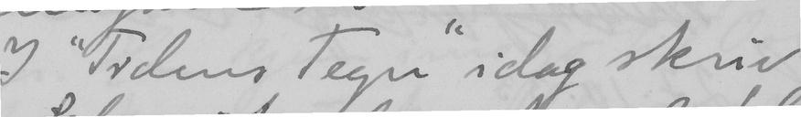I "Tidens Tegn" idag skriv
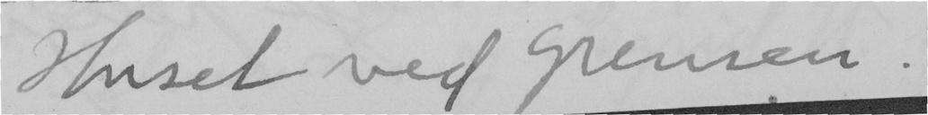"Huset ved Grensen".
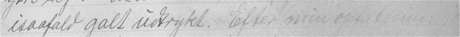isaafald galt udtrykt. Efter min opfatning: jo
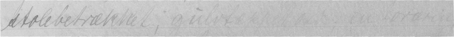stolebetrækket; gulvtæppet
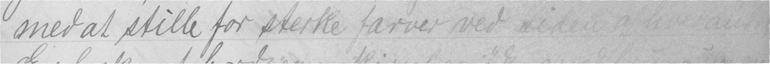med at stille for sterke farver ved siden af hverandre.
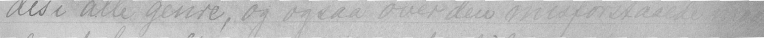des i alle genre, og ogsaa over den misforstaaede
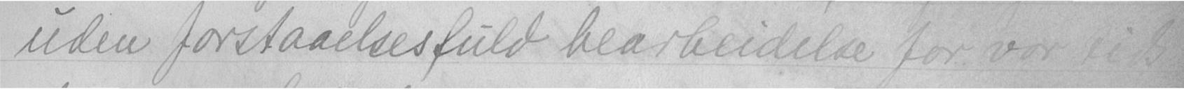uden forstaaelsesfuld bearbeidelse for vor tids
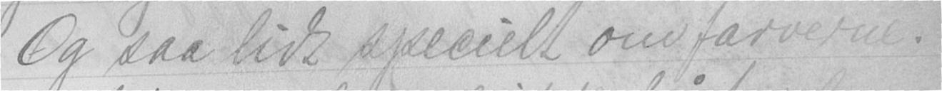Og saa lidt specielt om farverne.
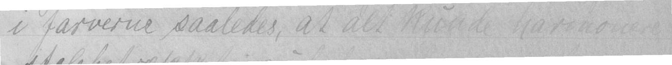i farverne saaledes, at det kunde harmonere med
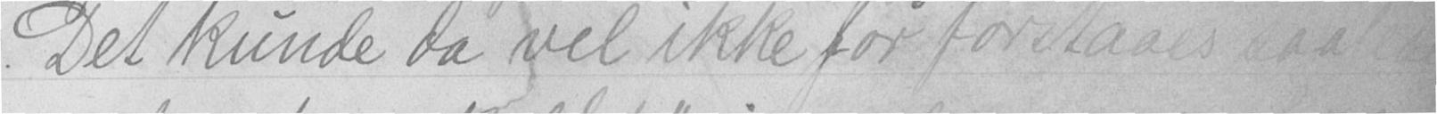Det kunde da vel ikke for forstaaes saaledes
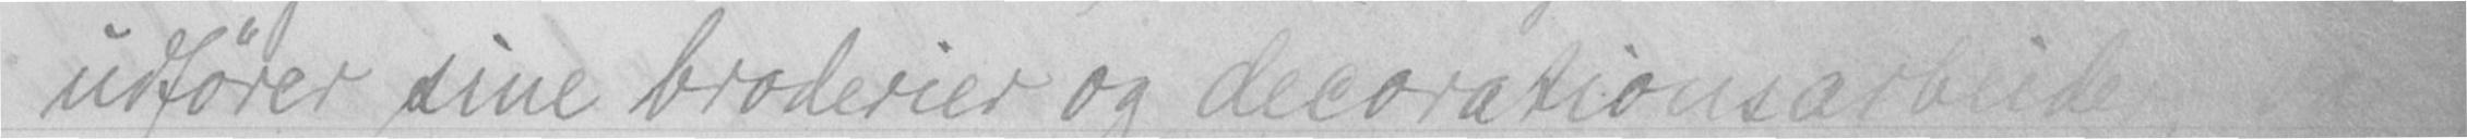udfører sine broderier og decorationsarbeider, var
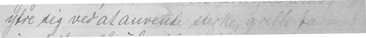ytre sig ved at anvende sterke, grelle farver? det var
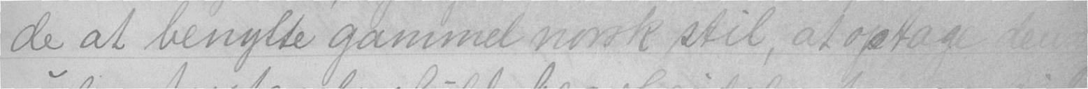de at benytte gammel norsk stil, at optage den
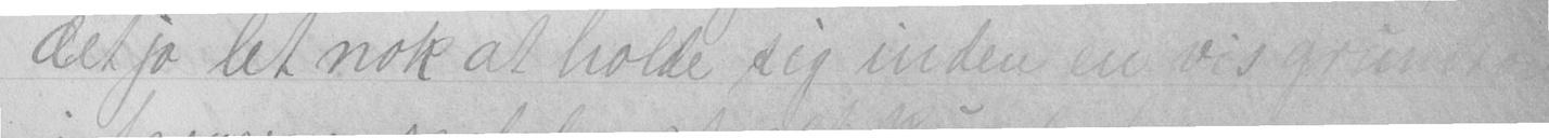det jo let nok at holde sig inden en vis grundtone
